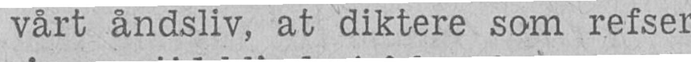vårt åndsliv, at diktere som refser
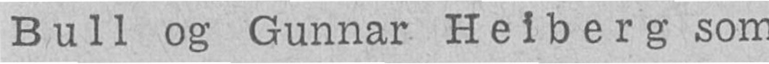Bull og Gunnar Heiberg som
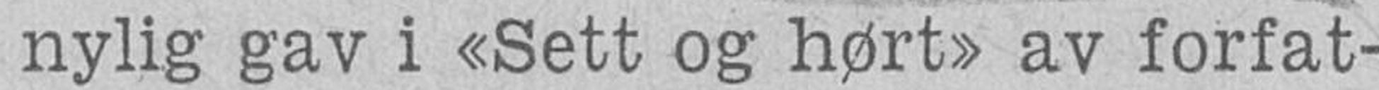nylig gav i "Sett og hørt" av forfat-
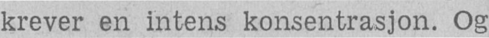krever en intens konsentrasjon. Og
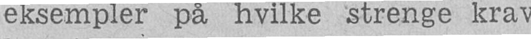eksempler på hvilke strenge krav
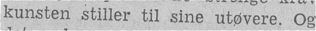kunsten stiller til sine utøvere. Og
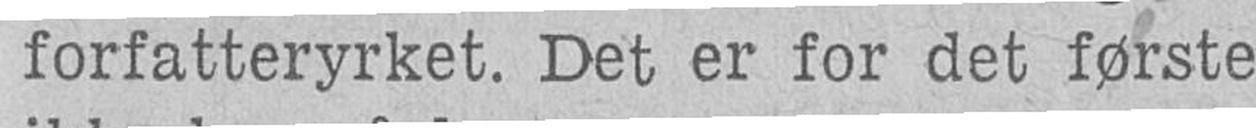forfatteryrket. Det er for det første
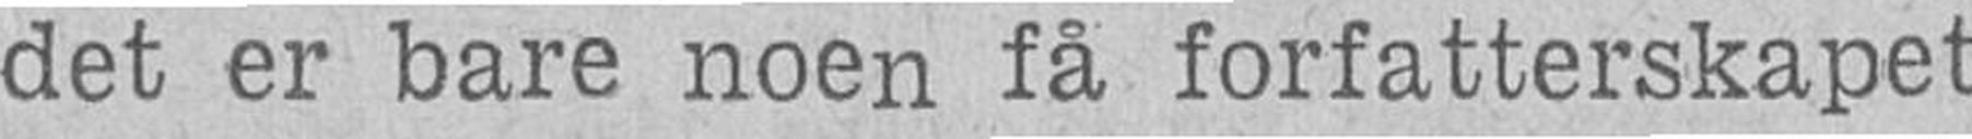det er bare noen få forfatterskapet
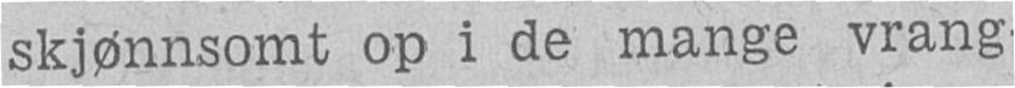skjønnsomt op i de mange vrang-
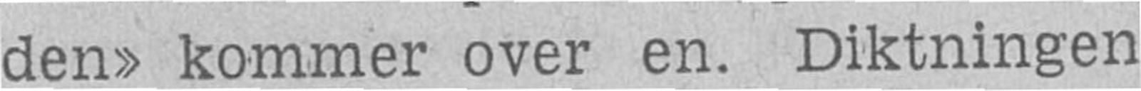den" kommer over en. Diktningen
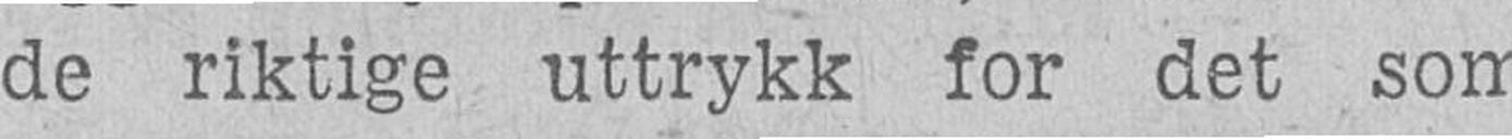de riktige uttrykk for det som
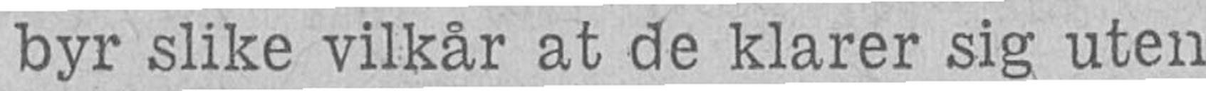byr slike vilkår at de klarer sig uten
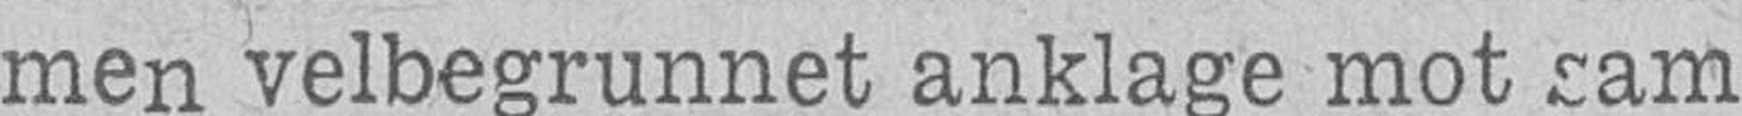men velbegrunnet anklage mot sam
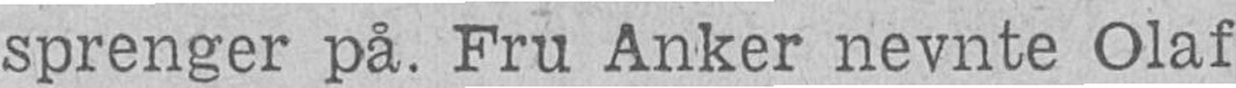sprenger pâ. Fru Anker nevnte Olaf
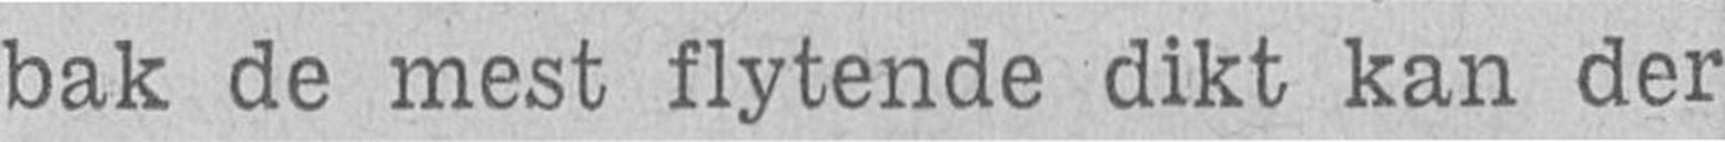bak de mest flytende dikt kan der
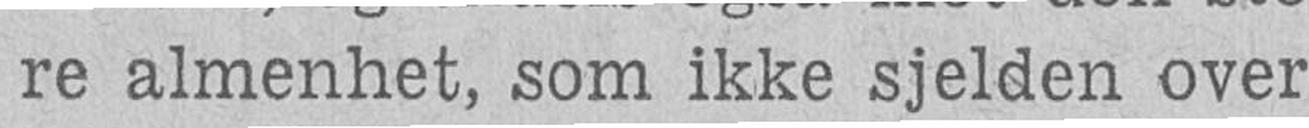re almenhet, som ikke sjelden over
